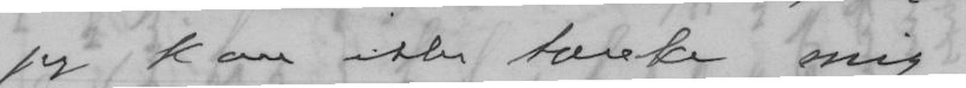jeg kan ikke tænke mig
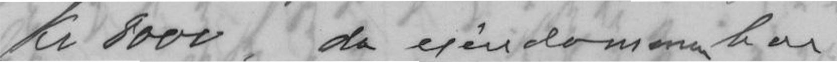kr 8000, da ejendommen har
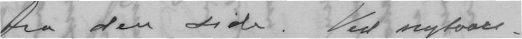fra den side. Ved nytaars-
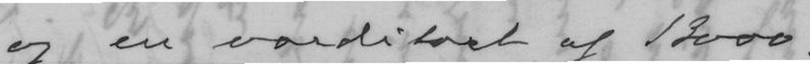og en værditaxt af 13000.
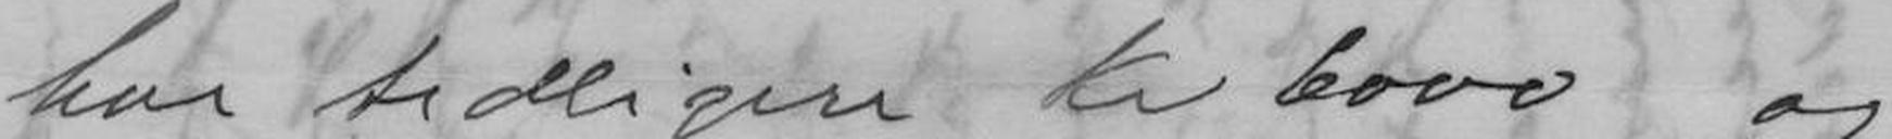har tidligere kr. 6000, og
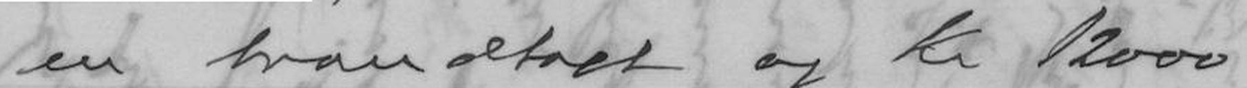en brandtaxt og kr 12000
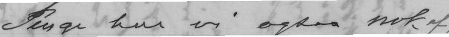Penge har vi ogsaa nok af,
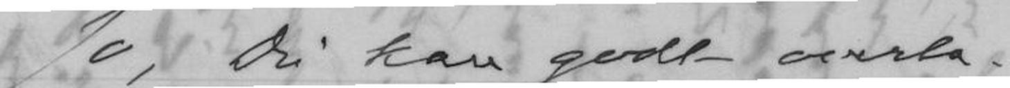Jo, Du kan godt overta-
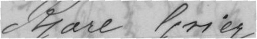Kjære Grieg!
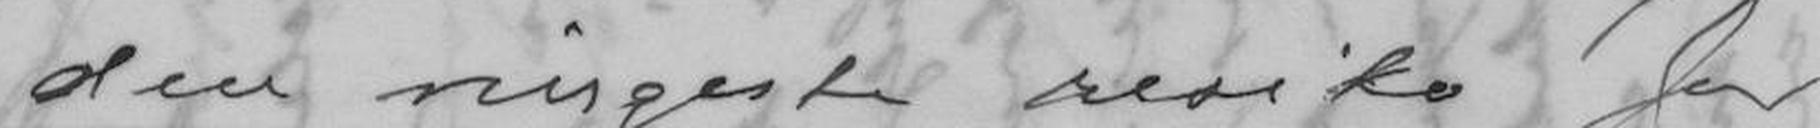den ringeste risiko for
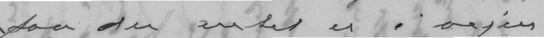saa den intet er i vejen
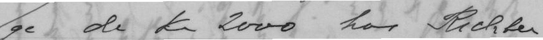ge de kr 2000 hos Richter,
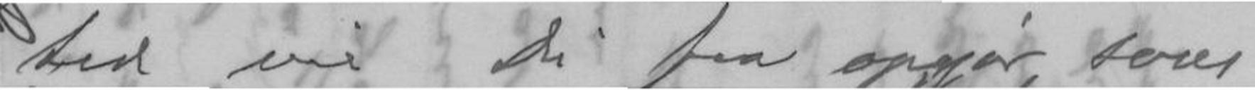tid vil Du faa opgjør, som
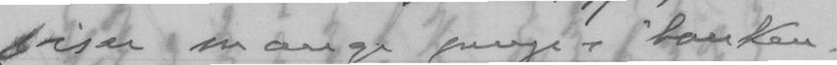viser mange penge i banken.
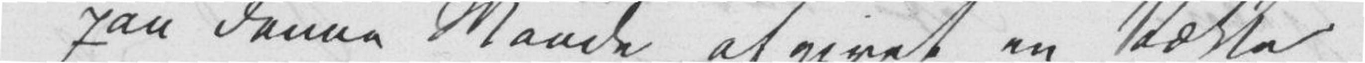paa denne Maade afgivet en Række
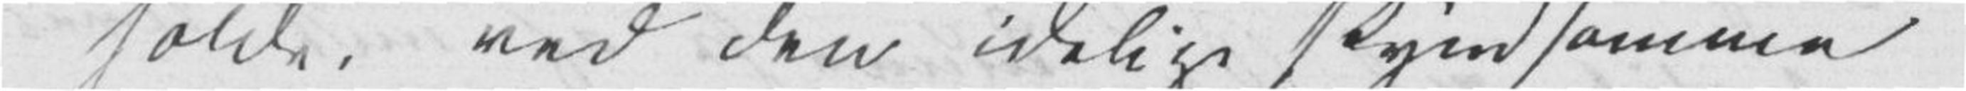holde, ved den idelige skyndsomme
